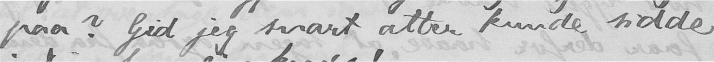paa? Gid jeg snart atter kunde sidde
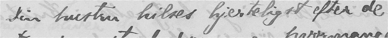Din hustru hilses hjerteligst efter de
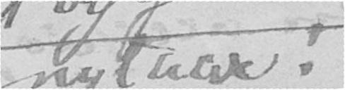nytaar!
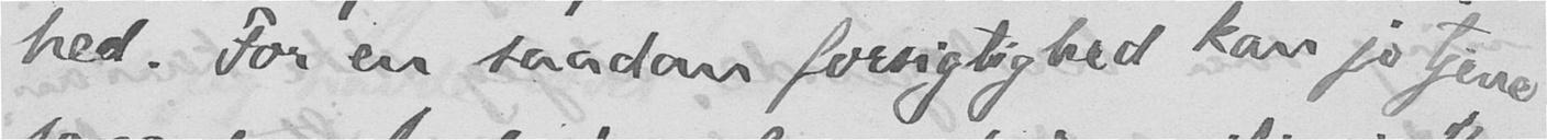hed. For en saadan forsigtighed kan jo tjene
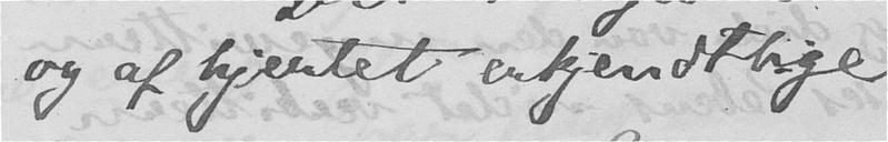og af hjertet erkjendtlige
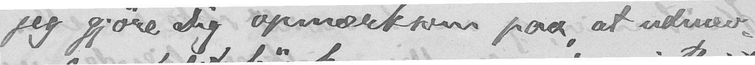jeg gjøre Dig opmærksom paa, at udnæv-
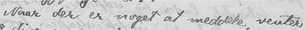Naar der er noget at meddele, venter
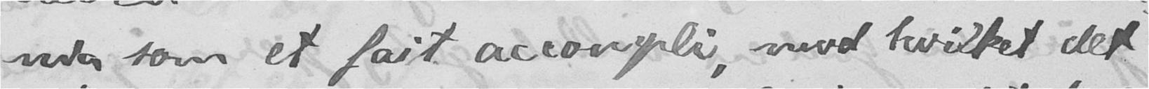nia som et fait accompli, mod hvilket det
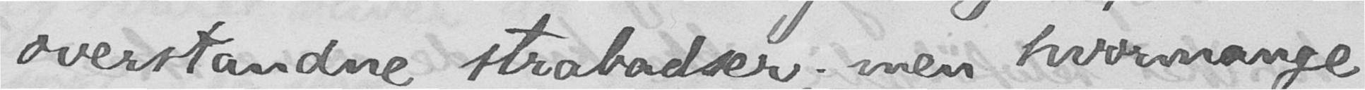overstandne strabadser; men hvormange
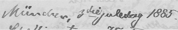München, 3de juledag 1885.
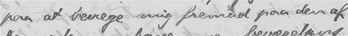paa at bevæge mig fremad paa den af
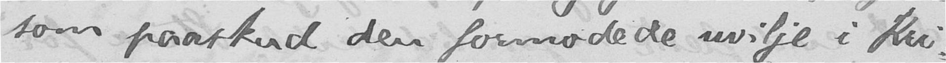som paaskud den formodede uvilje i Kri-
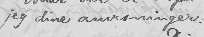jeg dine anvisninger.
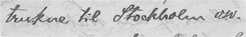trukne til Stockholm osv.
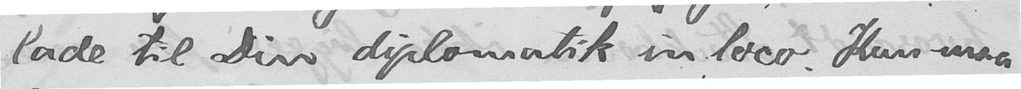lade til Din diplomatik in loco. Kun maa
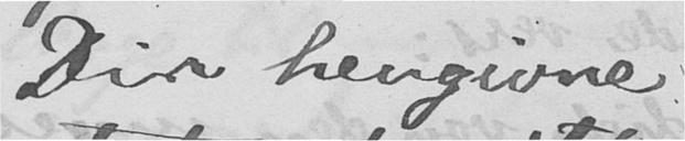Din hengivne
